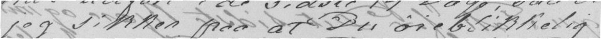jeg sikker paa at Du øieblikkelig
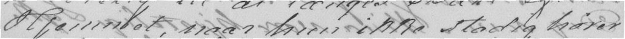Hjemmet, naar hun ikke stadig hører
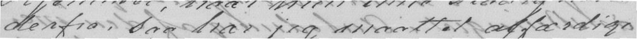derfra, saa har jeg maattet affærdige
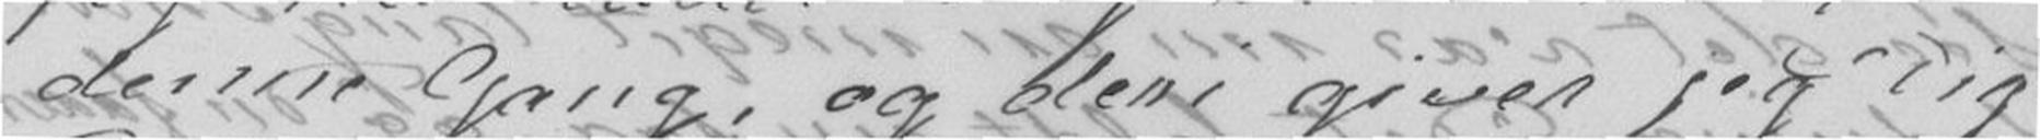denne Gang, og deri giver jeg Dig
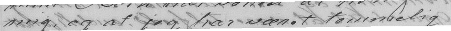mig, og at jeg har været temmelig
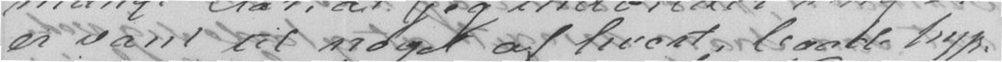er vant til noget af hvert lsaad hyp-
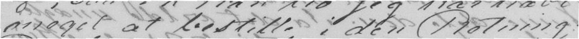meget at bestille i den Retning.
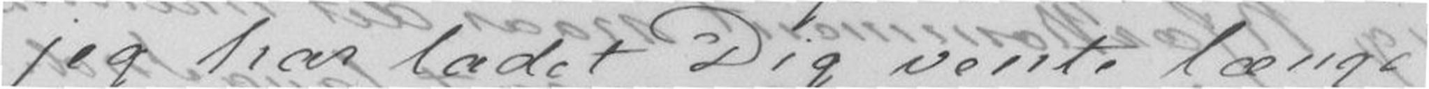jeg har ladet Dig vente længe
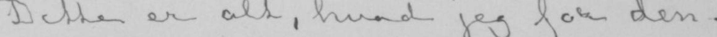Dette er alt, hvad jeg for den-
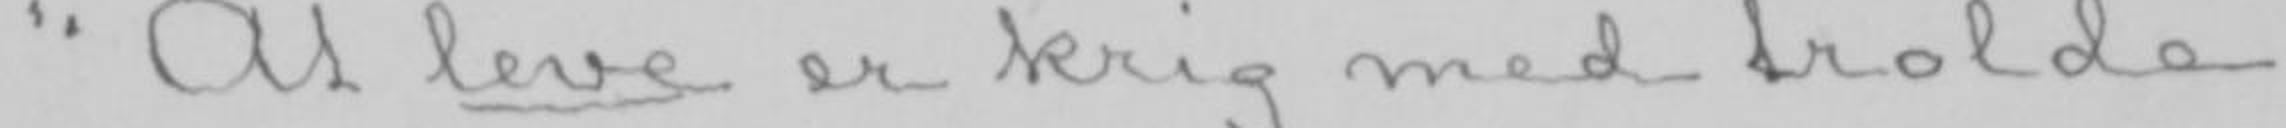«At leve er krig med trolde
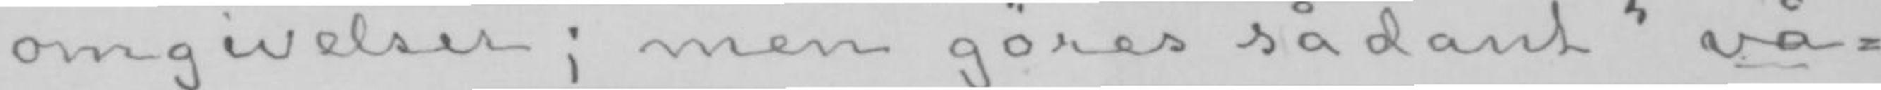omgivelser; men gøres sådant «vå-
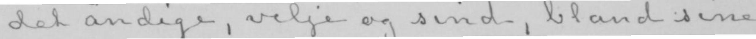det åndige, vilje og sind, bland sine
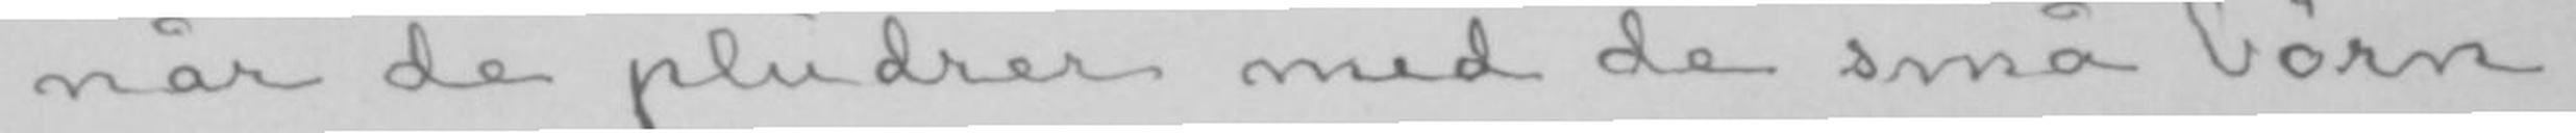når de pludrer med de små børn.
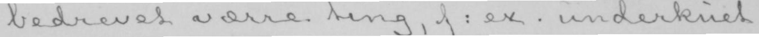bedrevet værre ting, f: ex. underkuet
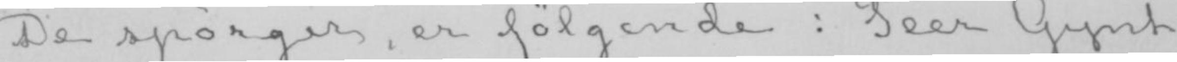De spørger, er følgende: Peer Gynt
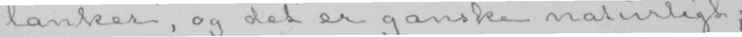lanker», og det er ganske naturligt;
